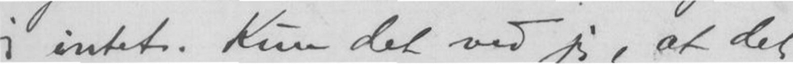jeg intet. Kun det ved jeg, at det
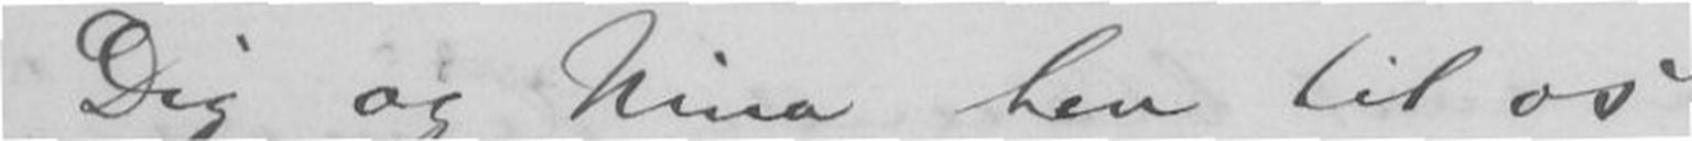Dig og Nina hen til os
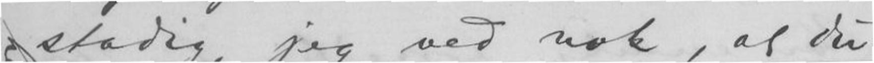stadig, jeg ved nok, at Du
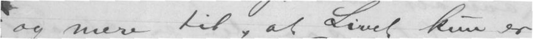og mere til, at Livet kun er
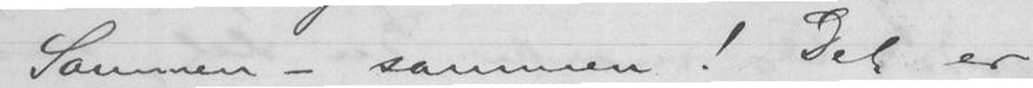Sammen - sammen! Det er
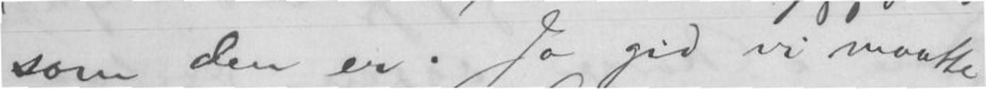som den er. Ja gid vi maatte
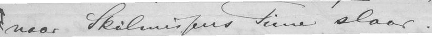naar Skilsmisens Time slaar.
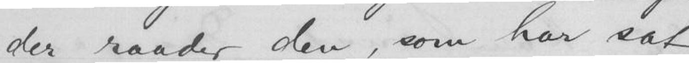der raader den, som har sat
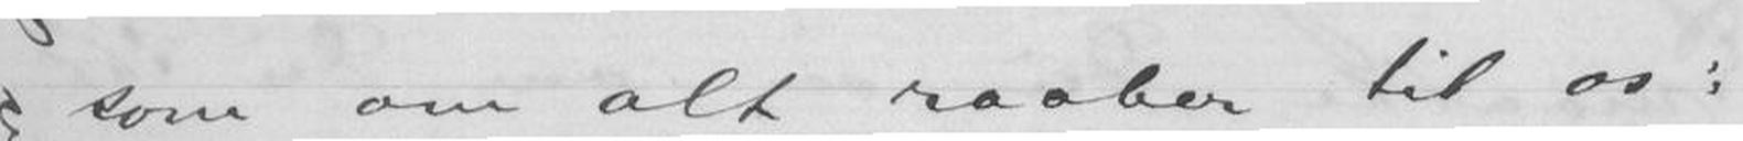som om alt raaber til os:
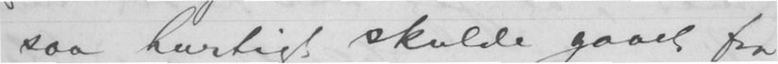saa hurtigt skulde gaaet fra
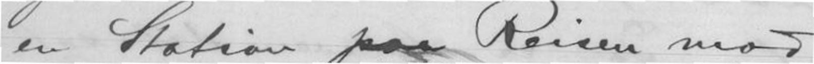en Station paa Reisen mod
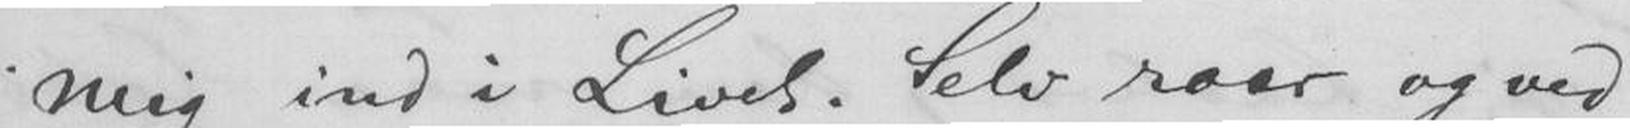mig ind i Livet. Selv raar og ved
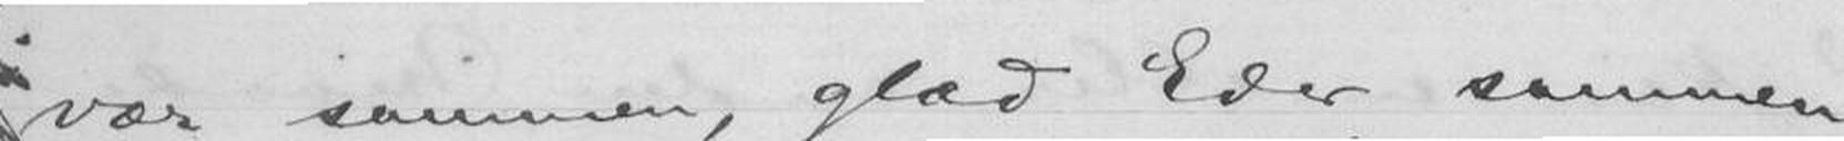vær sammen, glæd Eder sammen,
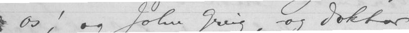os, og John Grieg, og Doktor
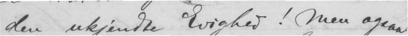den ukjendte Evighed! Men ogsaa
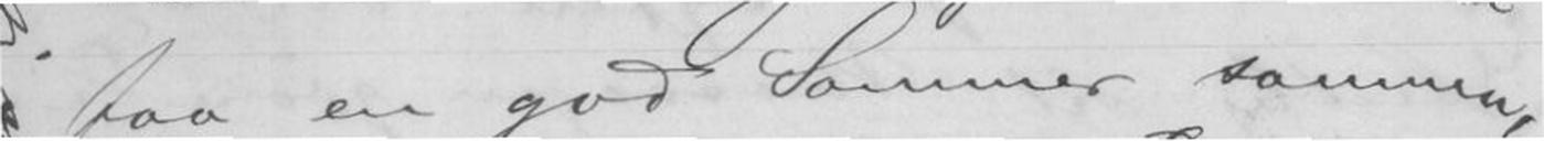faa en god Sommer sammen!
